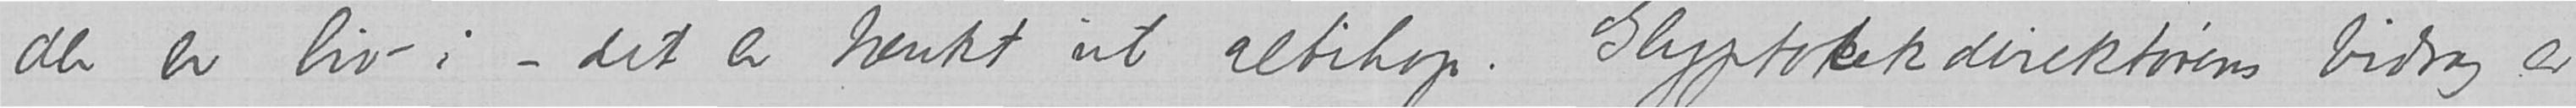der er liv i – det er tænkt ut altihop. Glyptotekdirektørens bidrag er
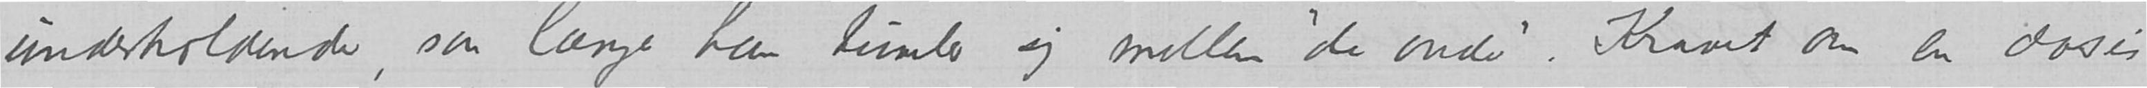underholdende, saa længe han tumler sig mellem «de onde». Kravet om en dosis
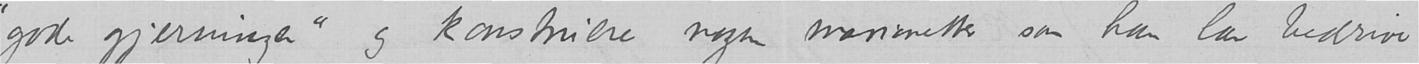«gode gjerninger» og konstruere nogen marionetter som han lar bedrive
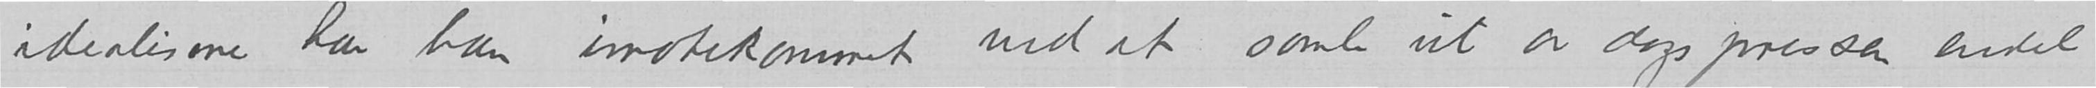idealisme har han imøtekommet med at samle ut av dagspressen endel
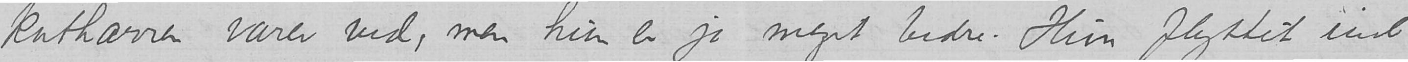katharren varer ved, men hun er jo meget bedre. Hun flyttet ind
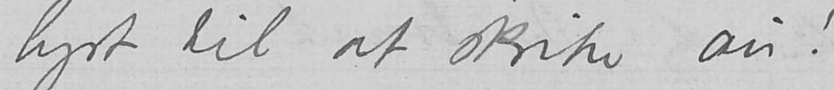lyst til at skrike au!
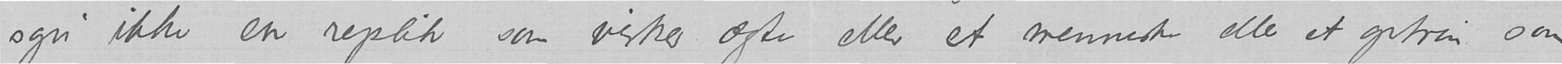sgu ikke en replik som virker ægte eller et menneske eller et optrin som
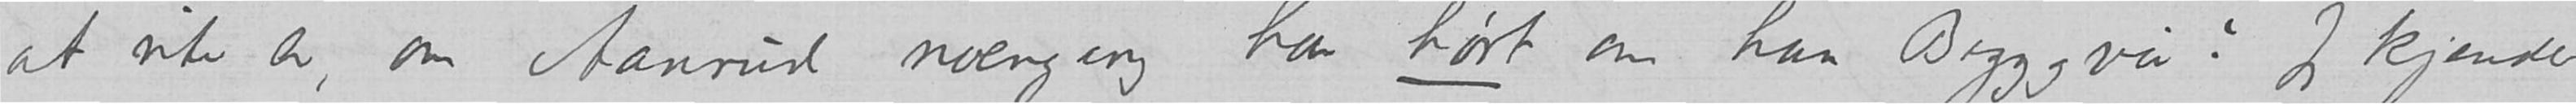at vite er, om Aanrud noengang har hørt om han Bryggvei? Jeg kjende
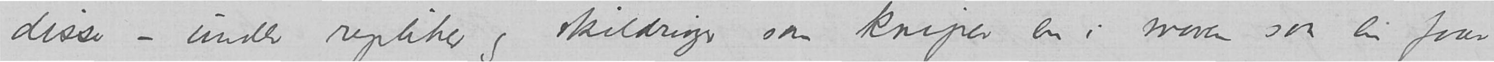disse – under repliker og skildringer som kniper en i maven saa ein faar
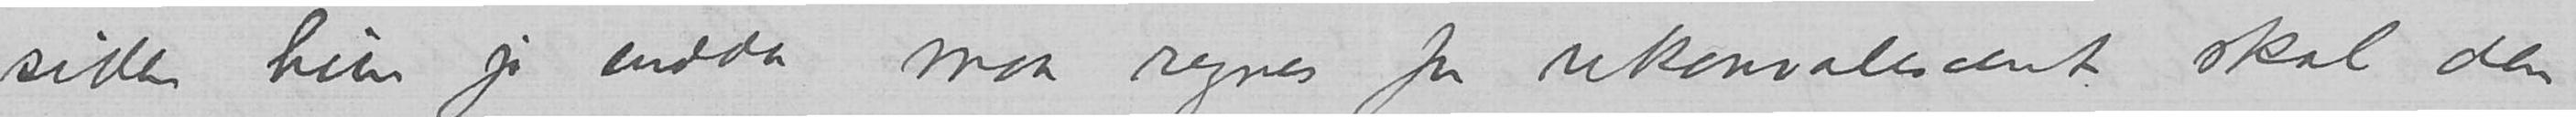siden hun jo endda maa regnes for rekonvalescent skal den
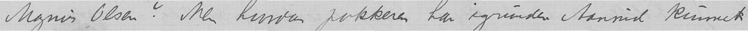Magnus Olsen? Men hvordan pokkeren har igrunden Aanrud kunnet
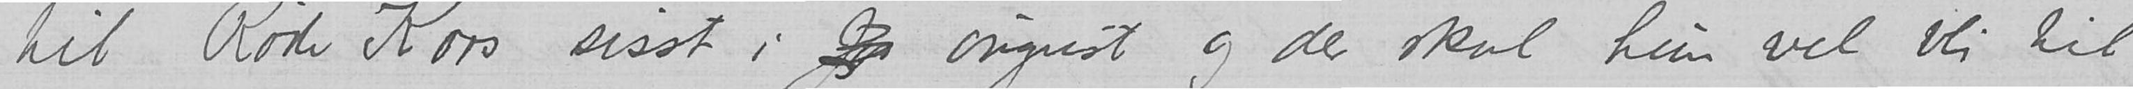til Røde Kors sisst i j august og der skal hun vel bli til
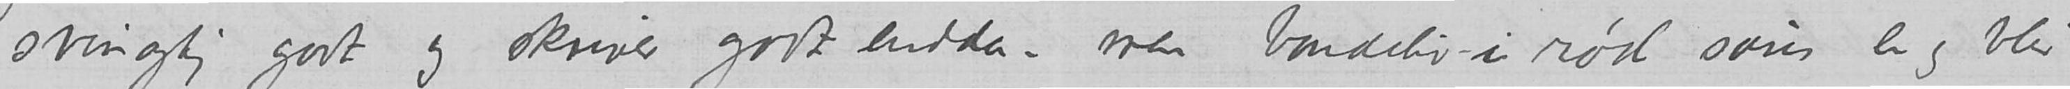svinagtig godt og skriver godt endda – men bondeliv i rød saus er og blir
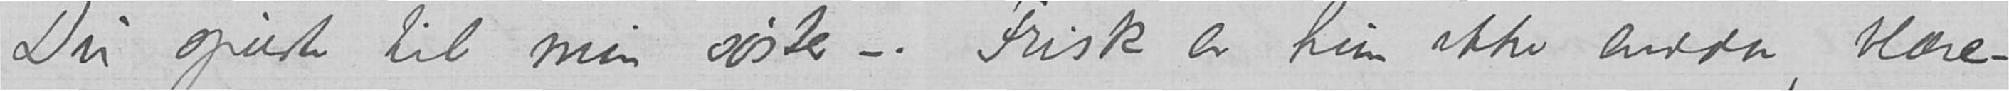Du spurte til min søster –. Frisk er hun ikke endda, blære-
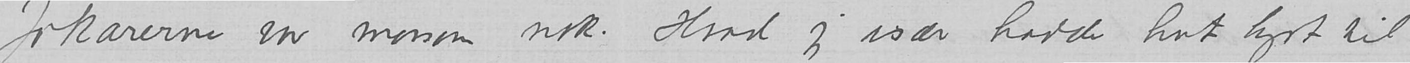Jokarerne er morsom nok. Hvad jeg især hadde hat lyst til
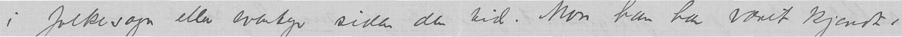i folkesagaen eller eventyr siden den tid. Mon han har været kjendt
